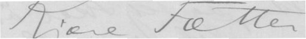Kjære Fætter
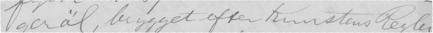gerøl, brygget efter Kunstens Regler
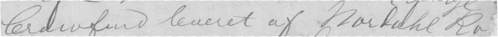Crawfend leveret af Nordahl Ro
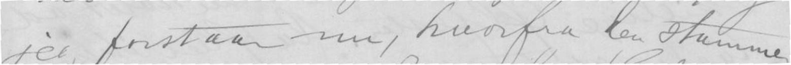jeg forstaar nu, hvorfra den stammer.
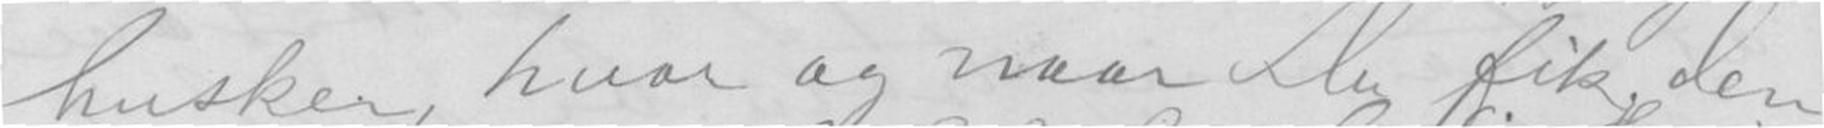husker, hvor og naar Du fik den,
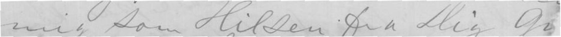mig som Hilsen fra Dig Gr
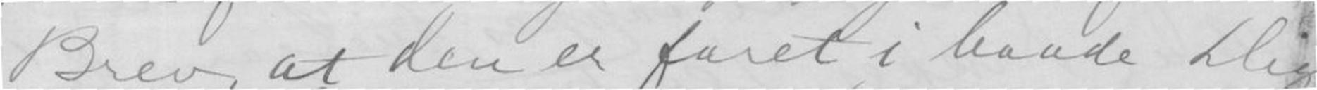Brev, at den er faret i baade Dig
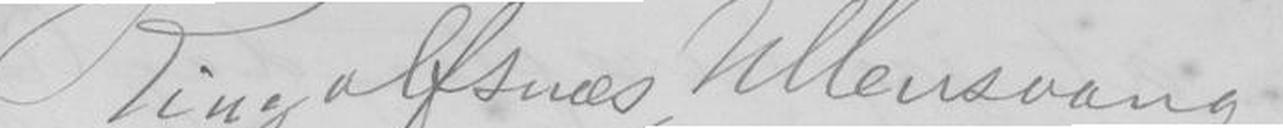Ringolfsnæs, Ullensvang
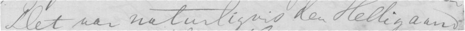Det var naturligvis den Helligaand,
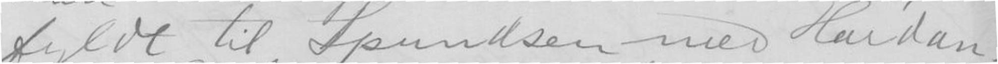fyldt til Spundsen med Hardan-
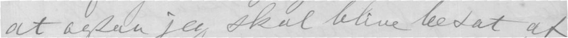at ogsaa jeg skal blive besat af
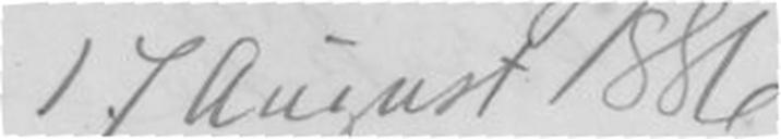17 August 1886.
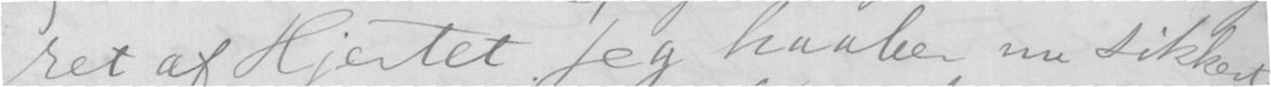ret af Hjertet jeg haaber nu sikkert
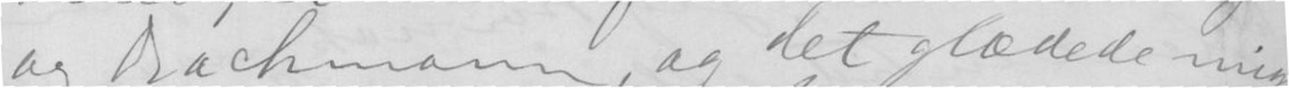og Drachmann, og det glædede mig
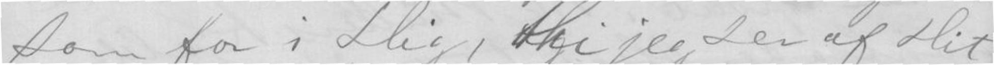som for i Dig, thi jeg ser af Dit
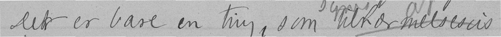Det er bare en ting, som tilnærmelsesvis
